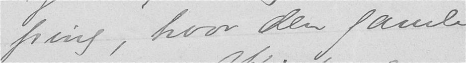ping, hvor den gamle
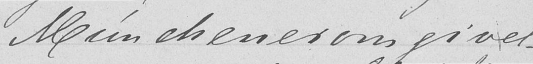Munchen er omgivel-
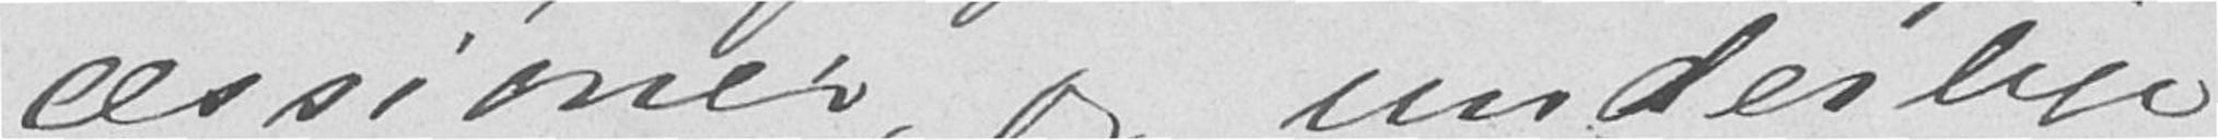cessioner, og underlige
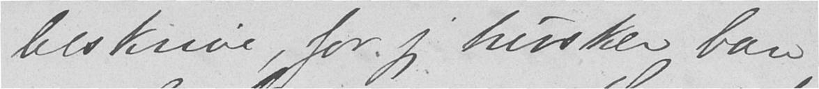beskrive, for jeg husker bare
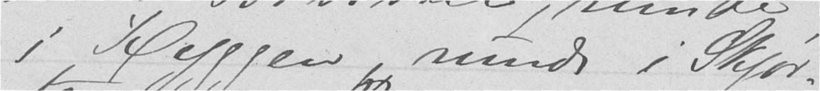i Ryggen, runde i Skjør-
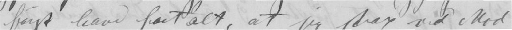først have fortalt, at jeg strax ved Mod-
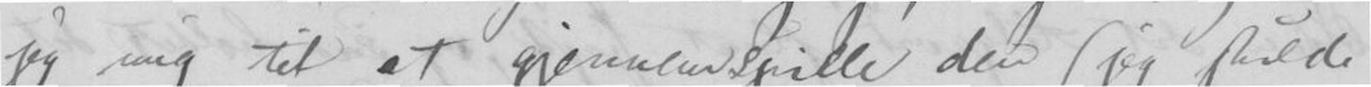jeg mig til at gjennemspille den (jeg skulde
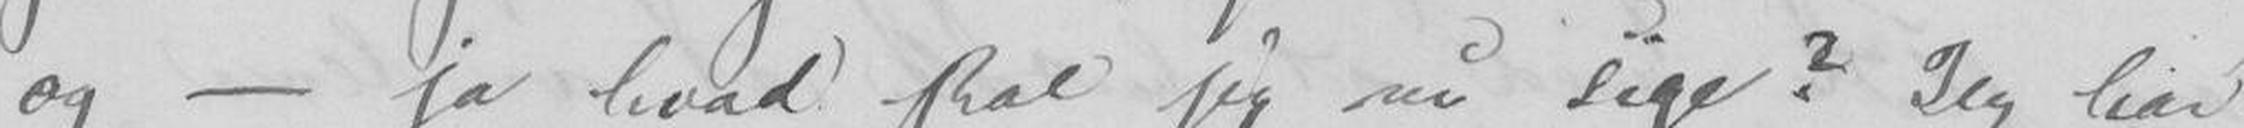og - ja hvad skal jeg nu sige? Jeg har
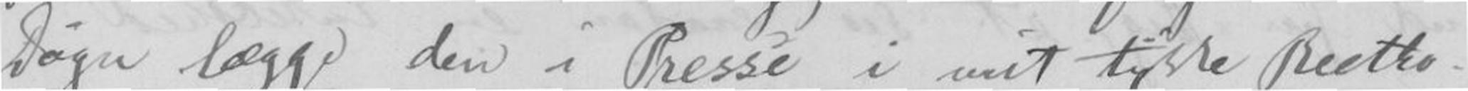Døgn lægge den i Presse i mit tykke Beetho-
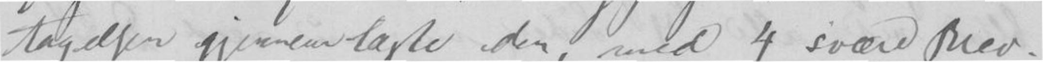tagelsen gjennemlagte den, med 4 svære Brev-
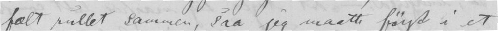fælt rullet sammen, saa jeg maatte først i et
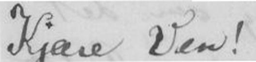Kjære Ven!
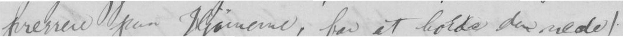presser paa Hjørnerne, for at holde den nede)
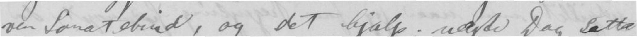ven Sonatebind, og det hjalp; næste Dag satte
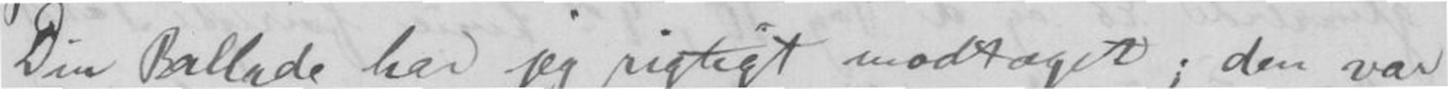Din Ballade har jeg rigtigt modtaget; den var
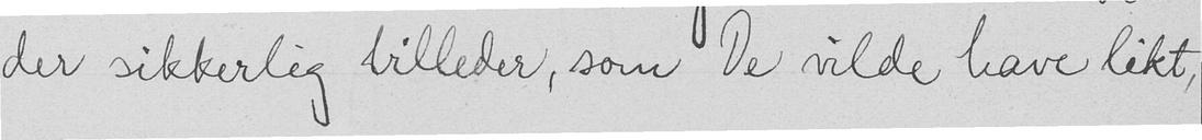der sikkerlig billeder, som De vilde have likt,
